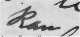kan
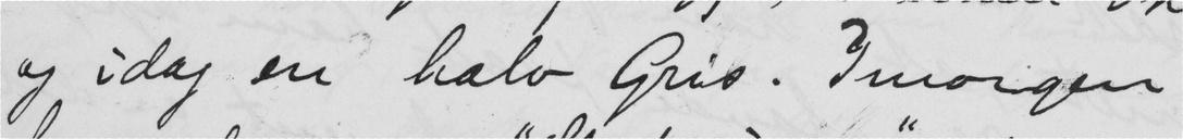og idag en halv Gris. Imorgen
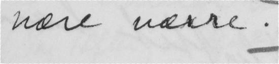være værre.
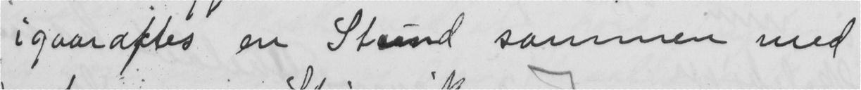igaaraftes en Stund sammen med
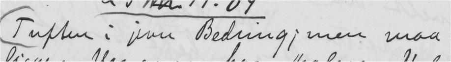Tuften i jevn Bedring; men maa
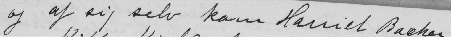og af sig selv kom Harriet Backer
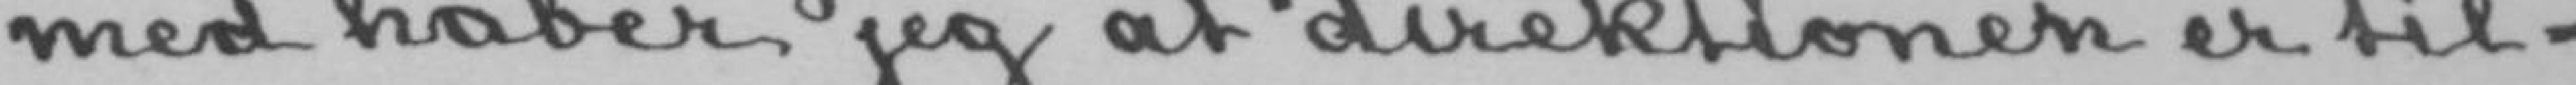med håber jeg at direktionen er til-
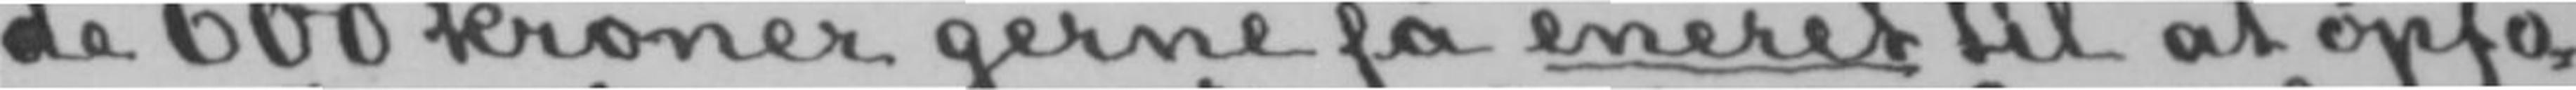de 600 kroner gerne få eneret til at opfø-
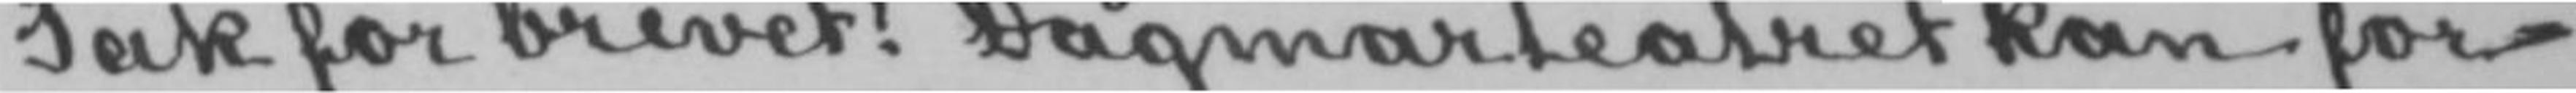Tak for brevet! Dagmarteatret kan for-
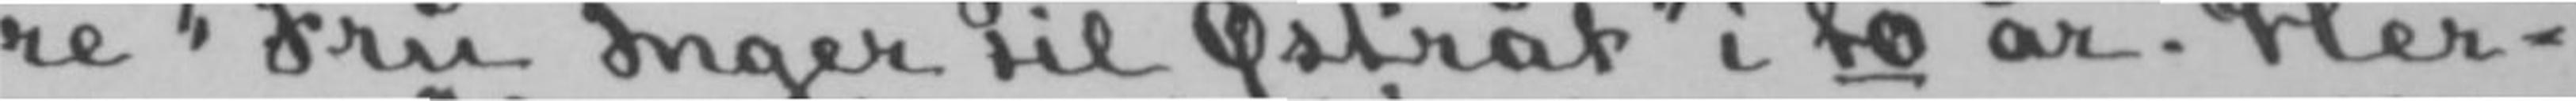re «Fru Inger til Østråt» i to år. Her-
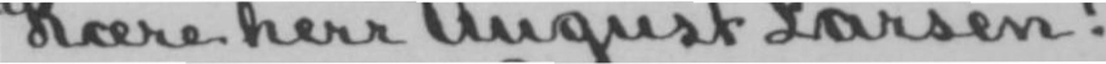Kære herr August Larsen!
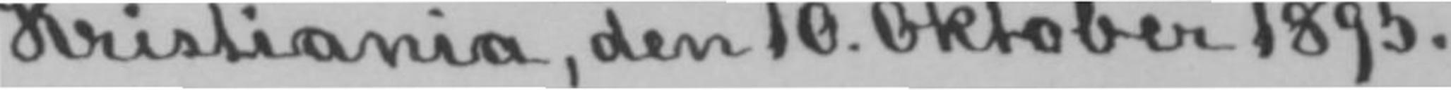Kristiania, den 10. Oktober 1895.
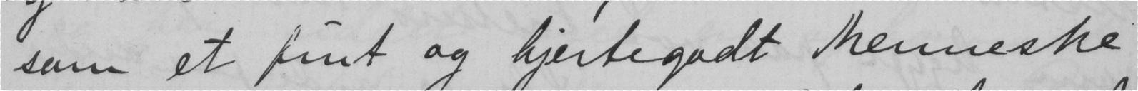som et fint og hjertegodt menneske
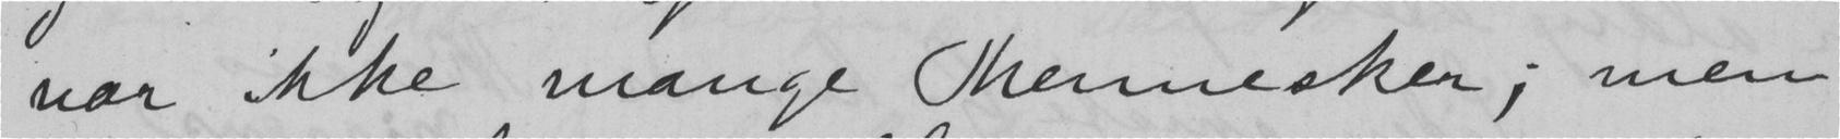var ikke mange mennesker; men
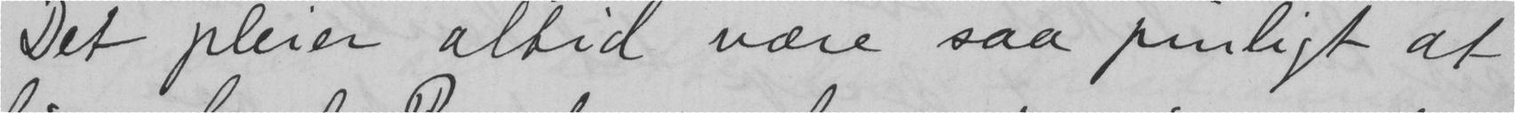Det pleier altid være saa pinligt at
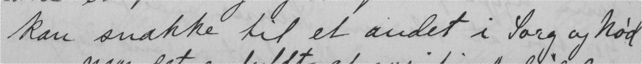kan snakke til et andet i Sorg og nød
